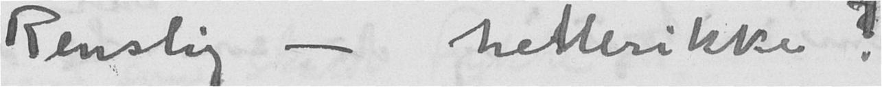Renslig - hellerikke!
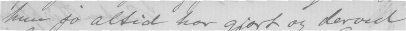hun jo altid har gjort og derved
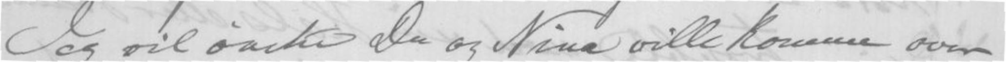Jeg vil ønske Du og Nina ville komme over
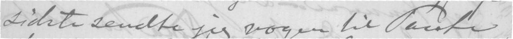sidste sendte jeg nogen til Tante
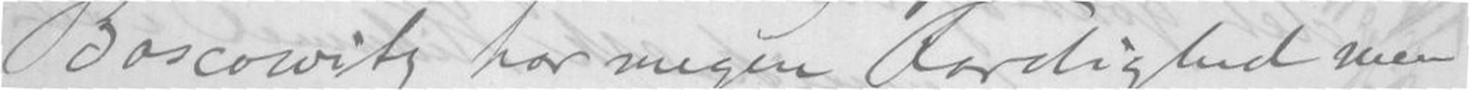Boscowitz har megen Fortrolighed men
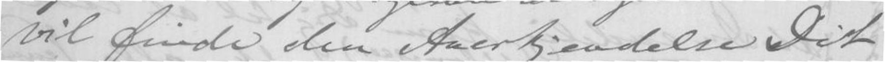vil finde den Anerkjendelse Dit
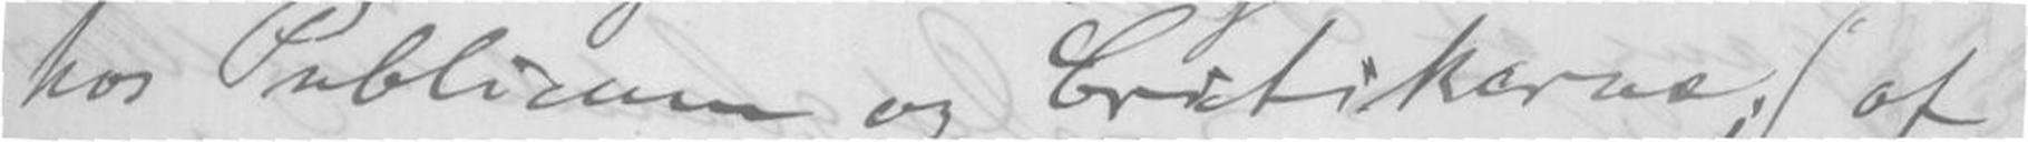hos Publicum og Critikerne; (af
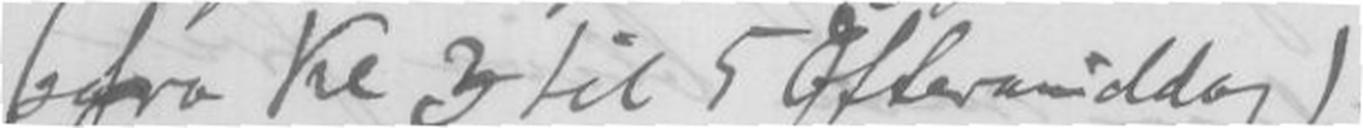(fra Kl 3 til 5 Eftermiddag)
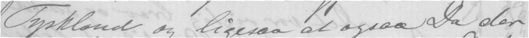Tyskland og ligesaa at ogsaa Du der
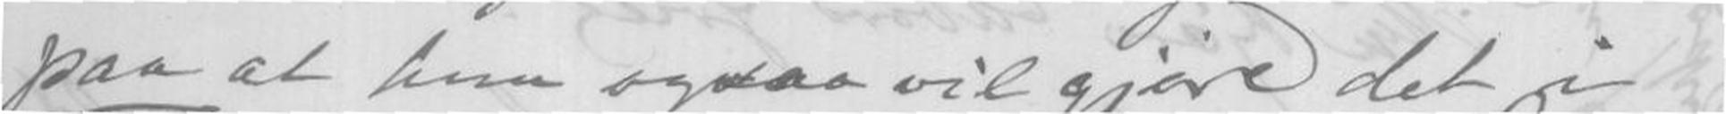paa at hun ogsaa vil gjøre det i
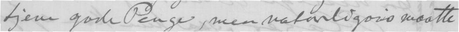tjene gode Penge, men naturligvis maatte
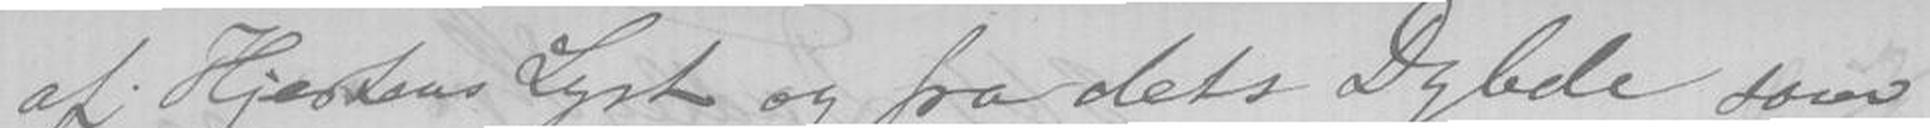af Hjertens Lyst og fra dets Dybde som
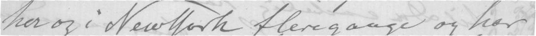her og i New York flere gange og har
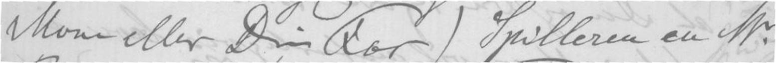Mone eller Din Far) Spilleren en Mr
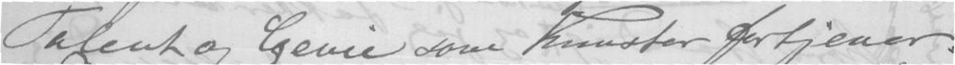Talent og Genie som Kunster fortjener.
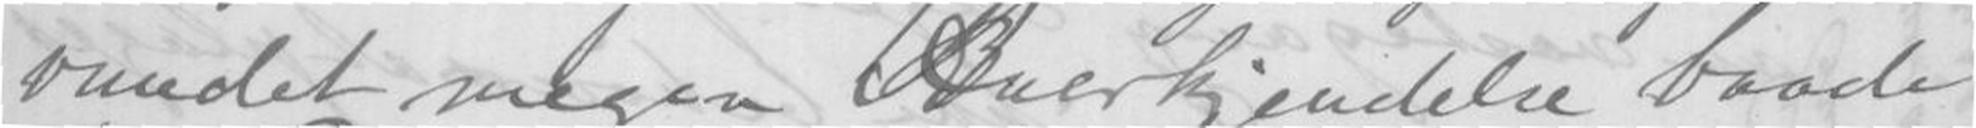vundet megen Anerkjendelse baade
